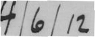4/6/12
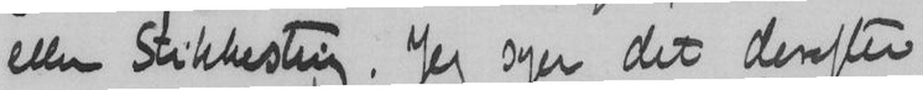eller Stilkesting. Jeg syer det derefter
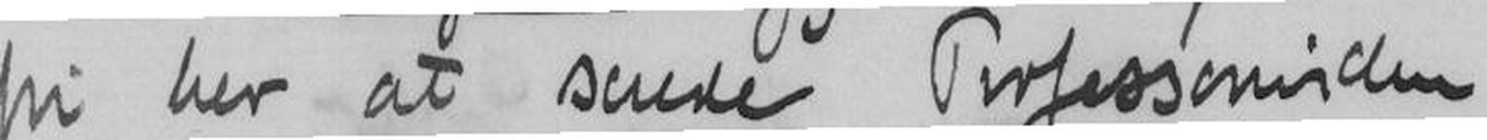fri her at sende Professorinden
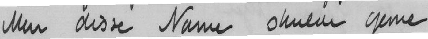Men disse Navn skulde gerne
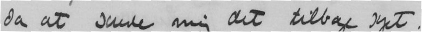da at sende mig det tilbage syet.
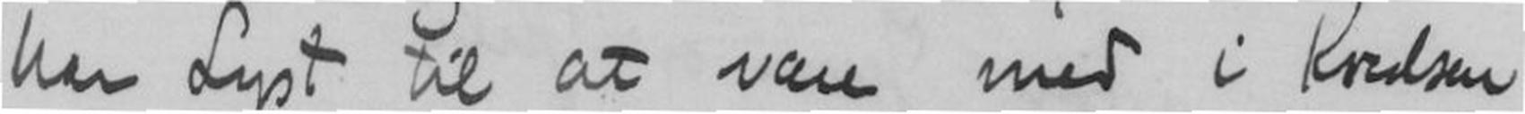har Lyst til at være med i Kredsen,
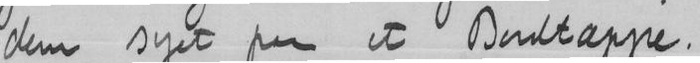dem syet paa et Bordtæppe.
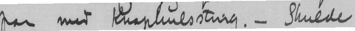paa med Knaphulsting. - Skulde
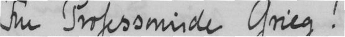Fru Professorinde Grieg!
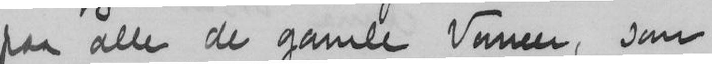paa alle de gamle Venner, som
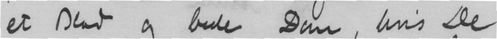et Blad og bede Dem, hvis De
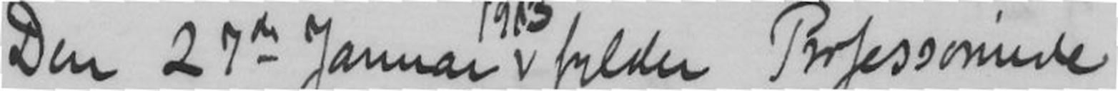Den 27de Januar fylder Professorinde
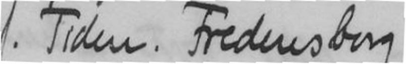f. Tiden Fredensborg.
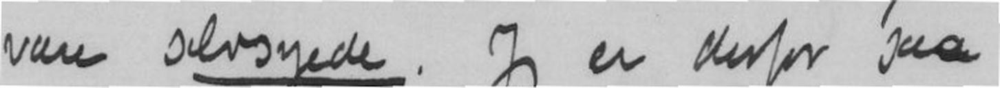være selvsyede. Jeg er derfor saa
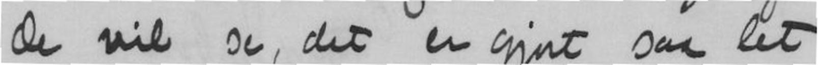De vil se, der er gjort saa let
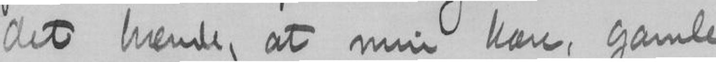det hende, at min kære, gamle
